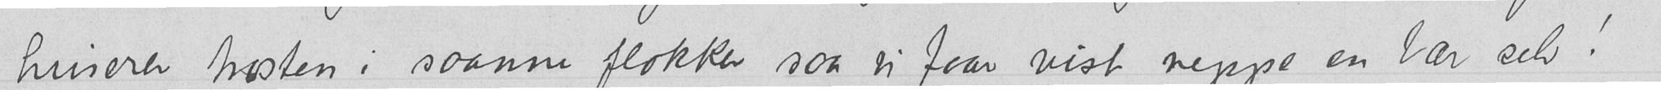huserer trosten i saanne flokker saa vi faar vist neppe en bær selv!
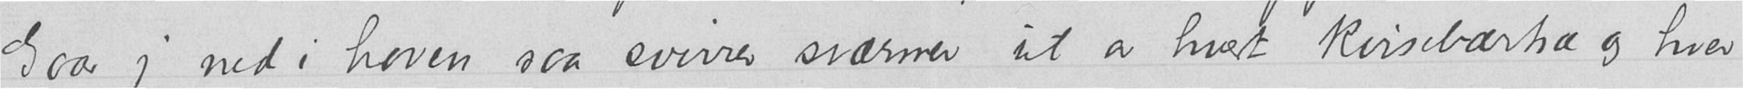Gaar jeg ned i haven saa svirrer sværmer ut av hvert kirsebærtræ og hver
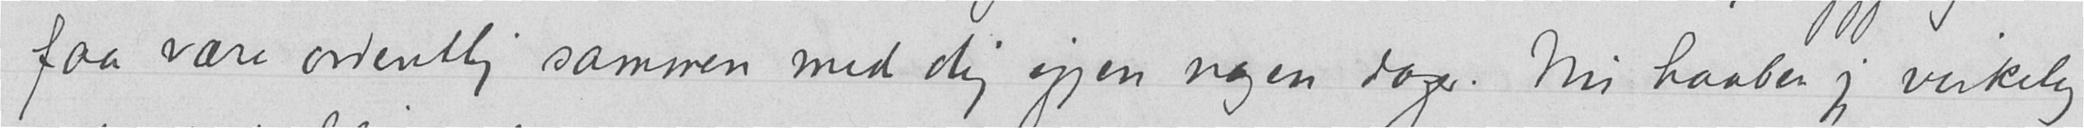faa være ordentlig sammen med dig igjen nogen dager. Nu haaber jeg virkelig
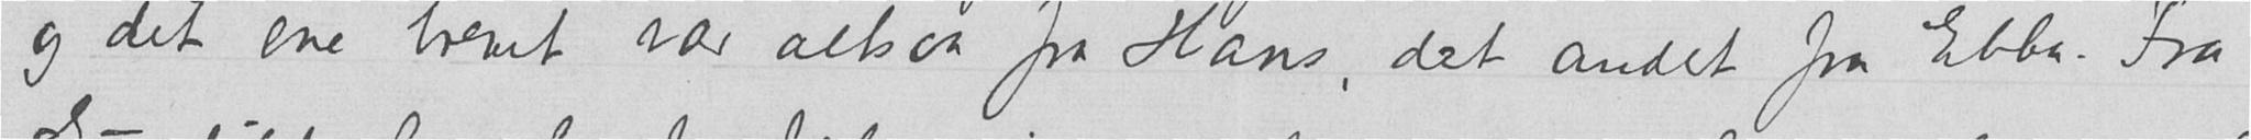og det ene brevet var altsaa fra Hans, det andet fra Ebba. Fra
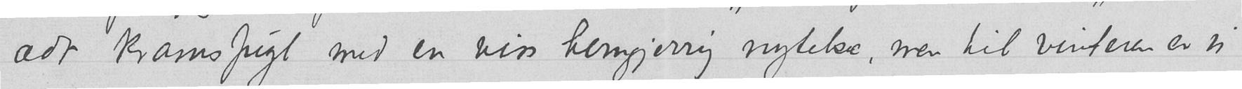ædt kramsfugl med en viss hevngjerrig nytelse, men til vinteren er vi
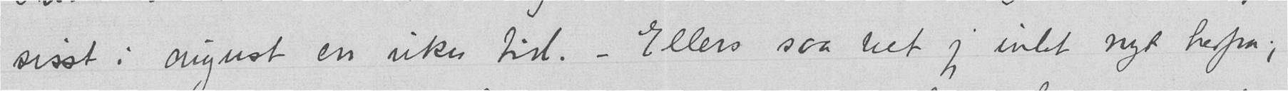sisst i august en ukes tid. – Ellers saa vet jeg intet nyt herfra;
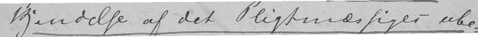kjendelse af det Pligtmæssiges ube-
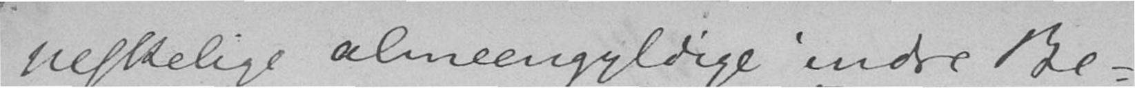neskelige almeengyldige indre Be-
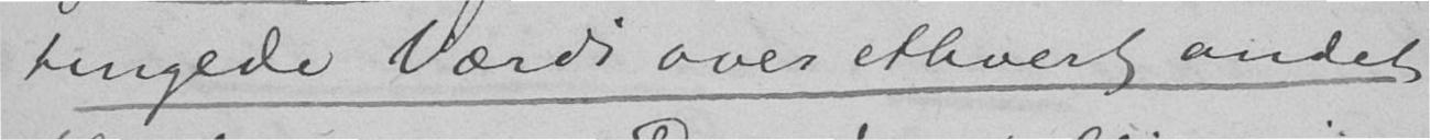tingede Værdi over ethvert andet
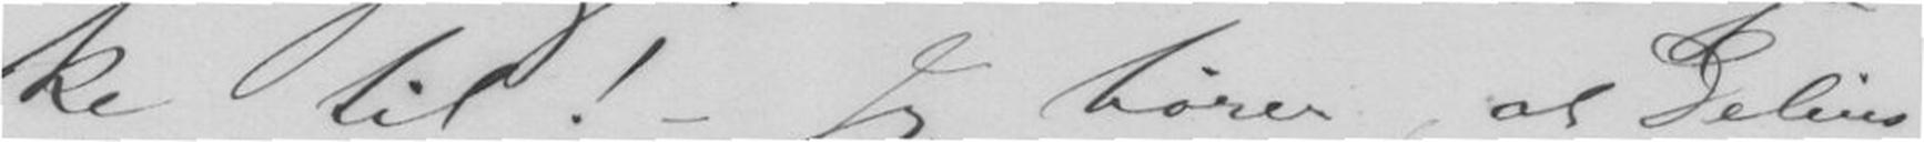ke til! - Jeg hører at Delius
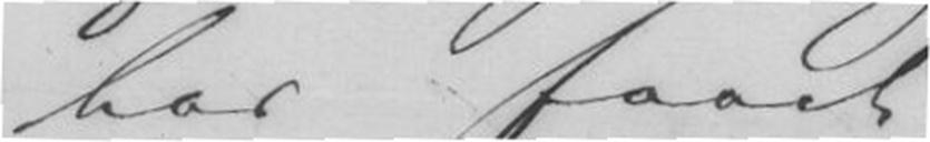har faaet
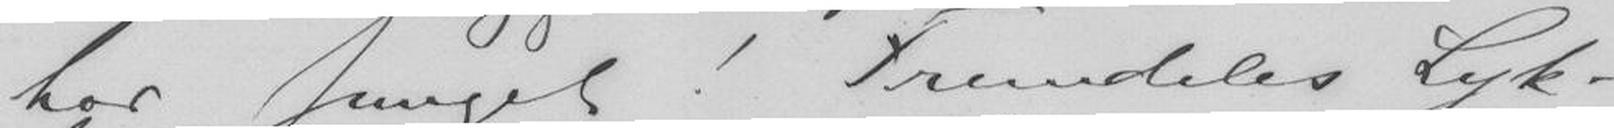har sunget! Fremdeles Lyk-
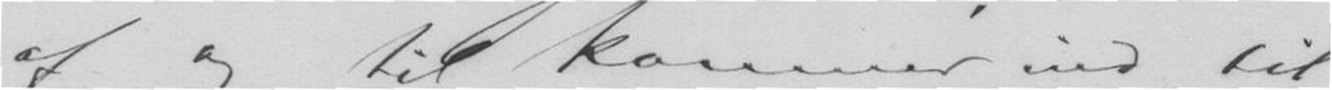af og til kommer ind til
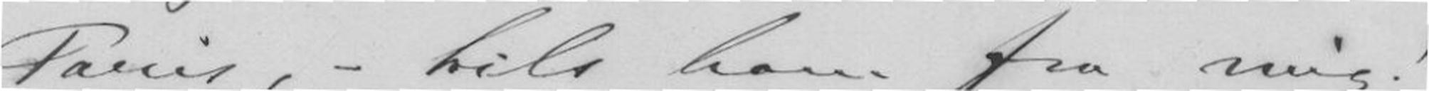Paris, - hils ham fra mig!
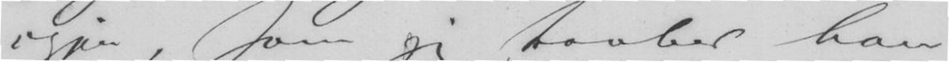igjen, som jeg haaber han
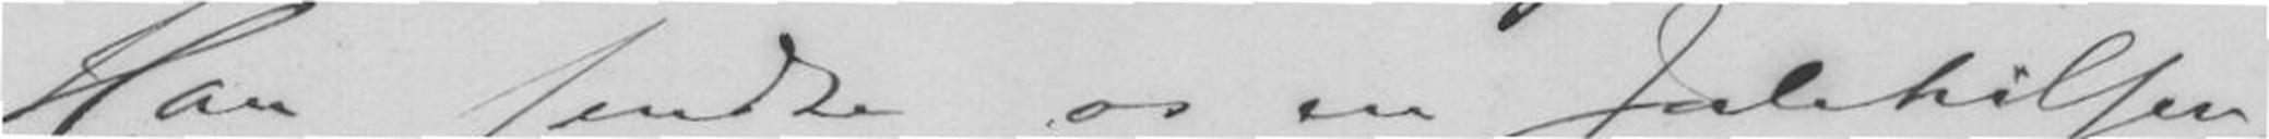Han sendte os en Julehilsen,
